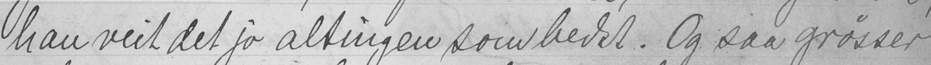han veit det jo altingen som bedst. Og saa grøsser
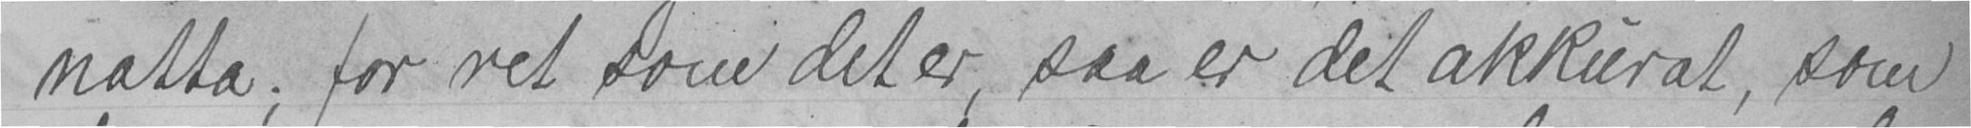natta; for ret som det er, saa er det akkurat, som
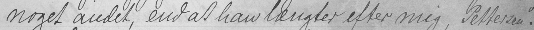noget andet, end at han længter efter mig, Peftersen".
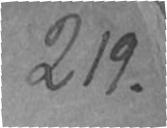219.
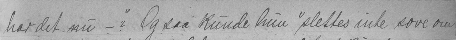har det nu - ?" Og saa kunde hun "slettes inte sove om
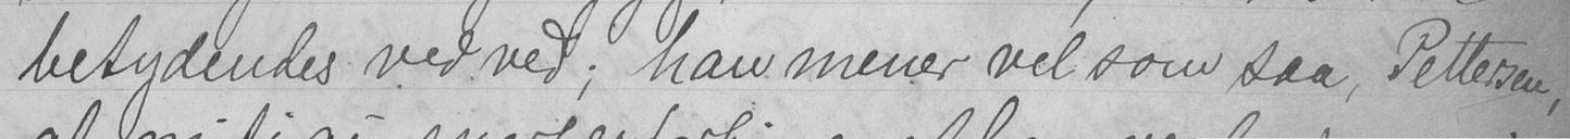betydendes ved ved; han mener vel som saa, Pettersen,
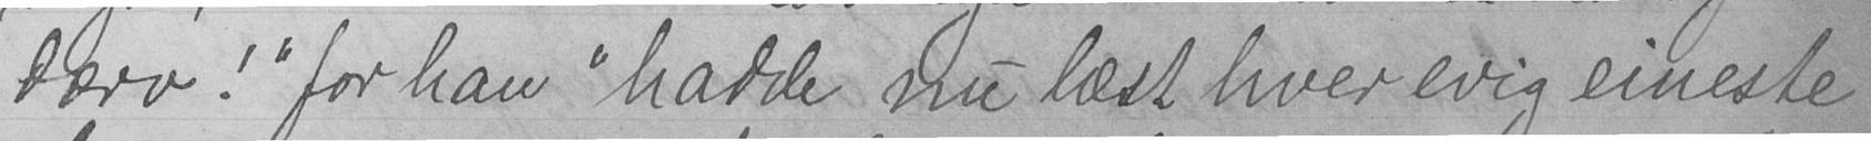dærv! "for han" hadde nu læst hver evig eineste
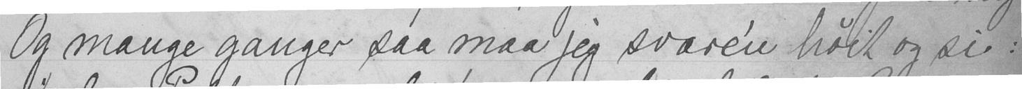Og mange ganger saa maa jeg svare'n høit og si:
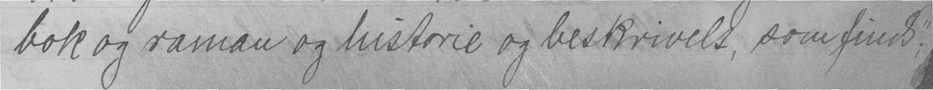bok og raman og historie og beskrivels, som finds;"
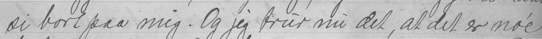si bort paa mig. Og jeg trur nu det, at det er no'e
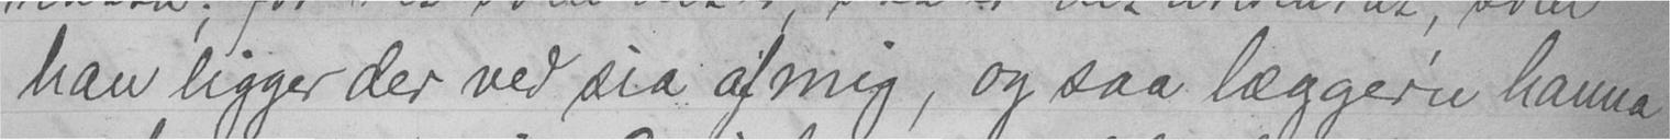han ligger der ved sia af mig, og saa lægger'n hanna
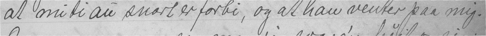at mi ti au snart er forbi, og at han venter paa mig.
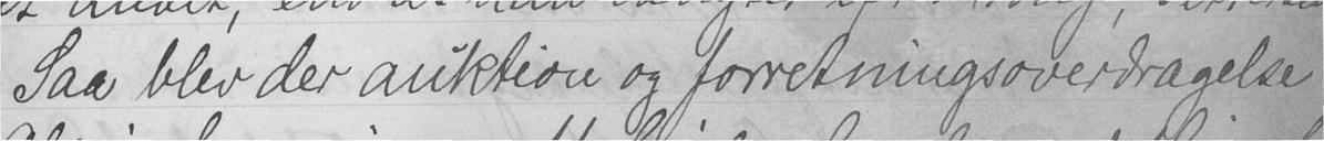Saa blev der auktion og forretningsoverdragelse
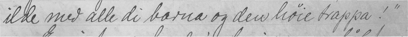ilde med alle di barna og den høie trappa!"
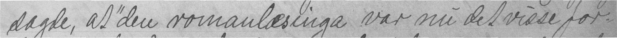sagde, at "den romanlæsinga var nu det visse for-
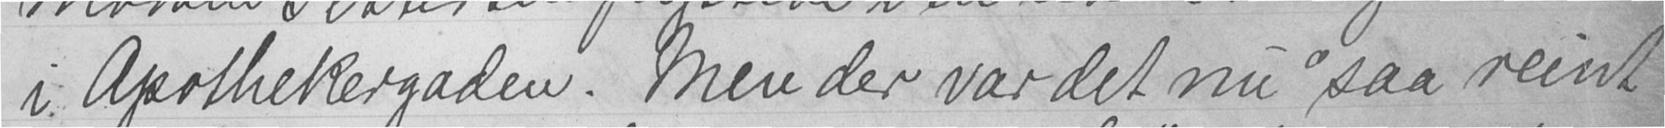i Apothekergaden. Men der var det nu "saa reint
